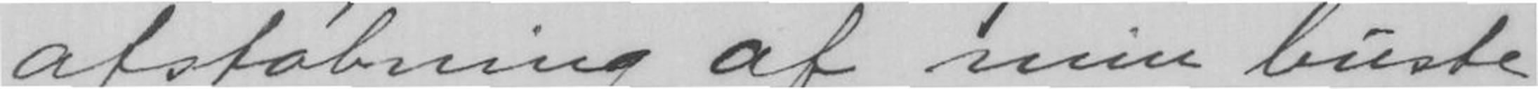afstøbning af min buste
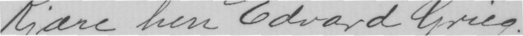Kjære herr Edvard Grieg.
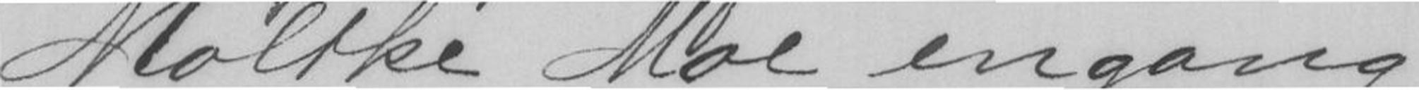Moldke Moe engang
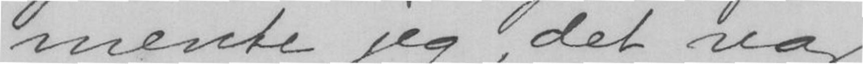mente jeg, det var
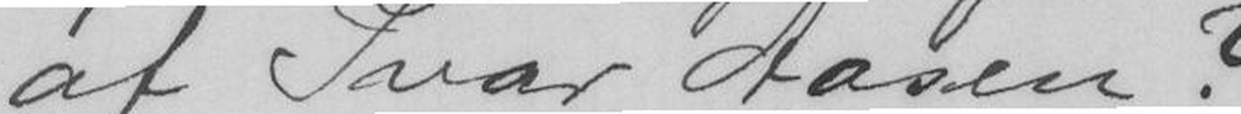af Ivar Aasen?
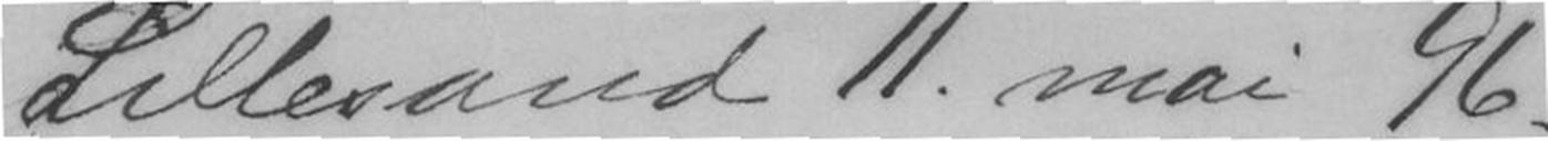Lillesand 11 mai 96.
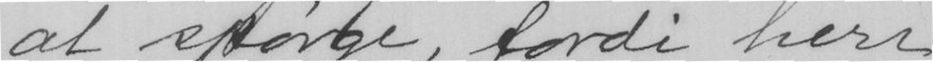at spørge, fordi herr
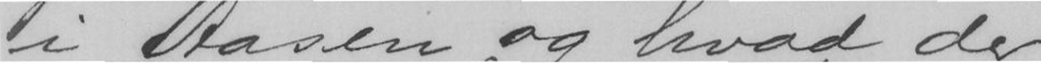i Aasen og hvad der
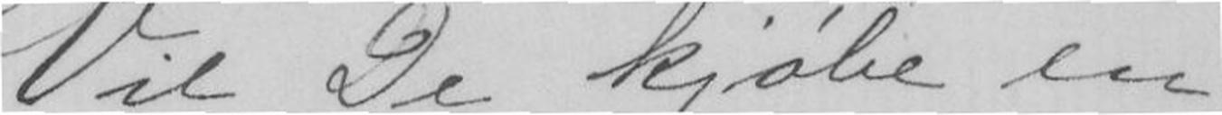Vil De kjøbe en
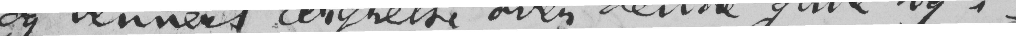og venners ærgrelse over denne gave tog i-
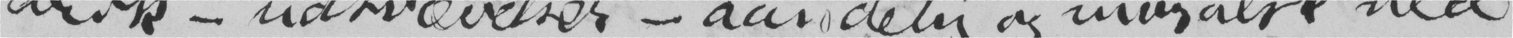drik – udsvævelser – aandelig og moralsk ned-
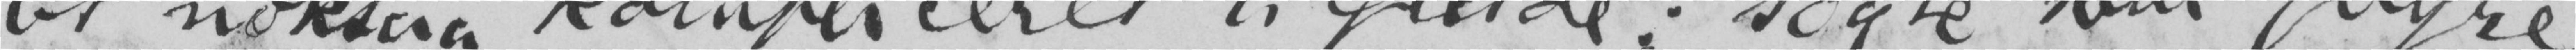Et noksaa kompliceret tilfælde: søgte som yngre
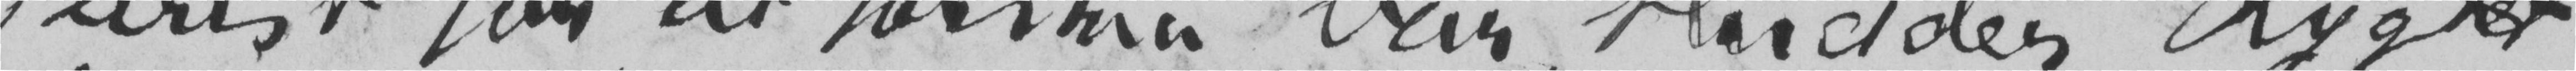jurist for at forstaa var sludder. Rygtet
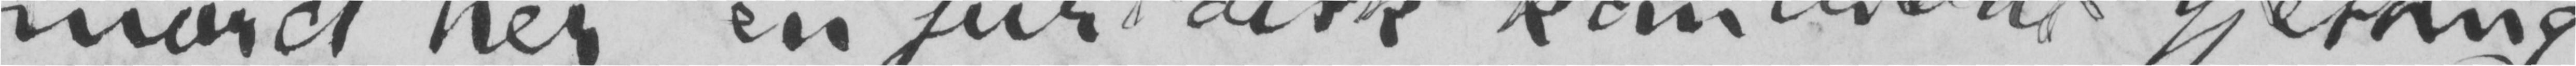mord her, en juridisk kandidat Gjessing,
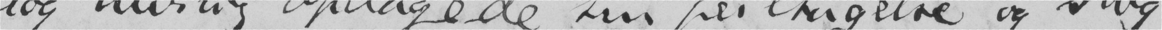dog hurtig opdagede sin feiltagelse og slog
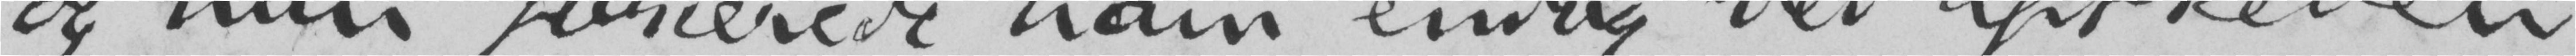og hun forærede ham endog ved afskeden
